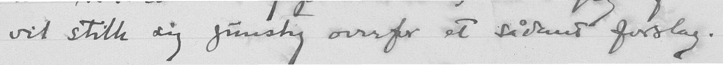vil stille sig gunstig overfor et sådant forslag.
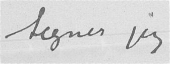tegner jeg
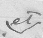et
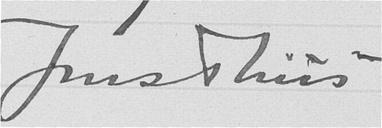Jens Thiis
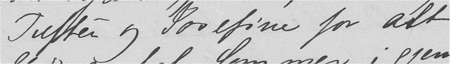Tuften og Josefine for alt
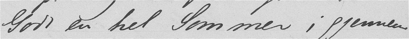Godt en hel Sommer igjennem
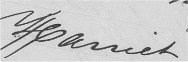Harriet
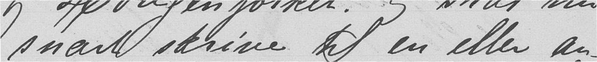snart skrive til en eller an-
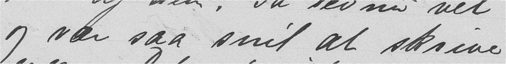og vær saa snil at skrive
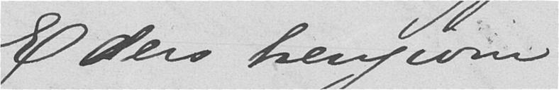Eders hengivne
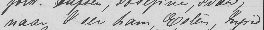naar I ser ham, Ester, Ingrid
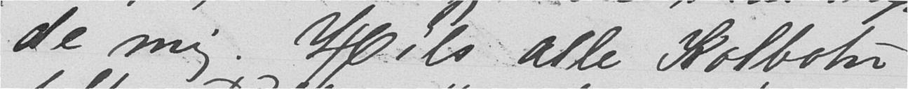de mig. Hils alle Kolbotn-
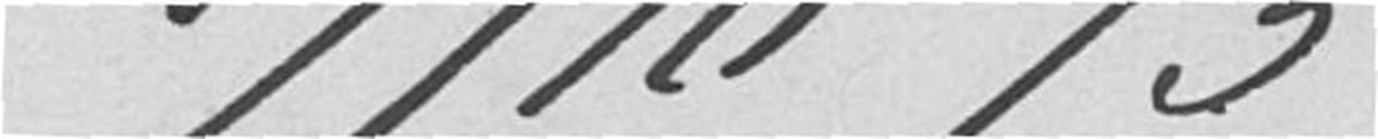19/10 93
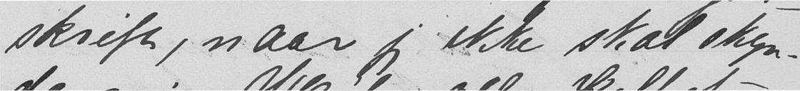skrift, naar jeg ikke skal skyn-
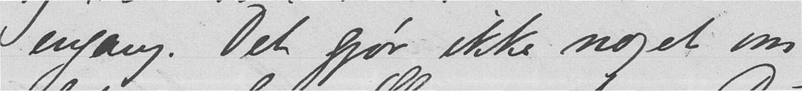engang. Det gjør ikke noget om
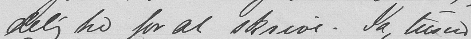delighed for at skrive. Ja, tusind
